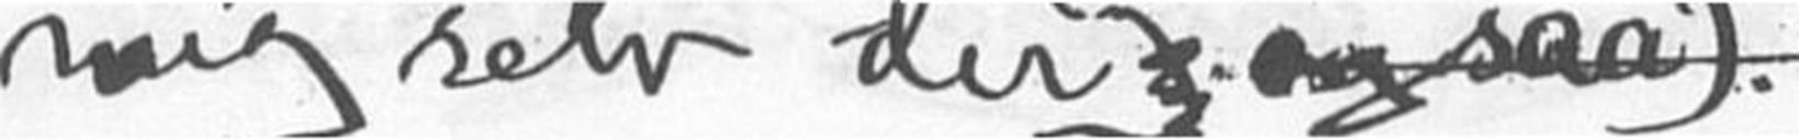mig selv der ogsaa).
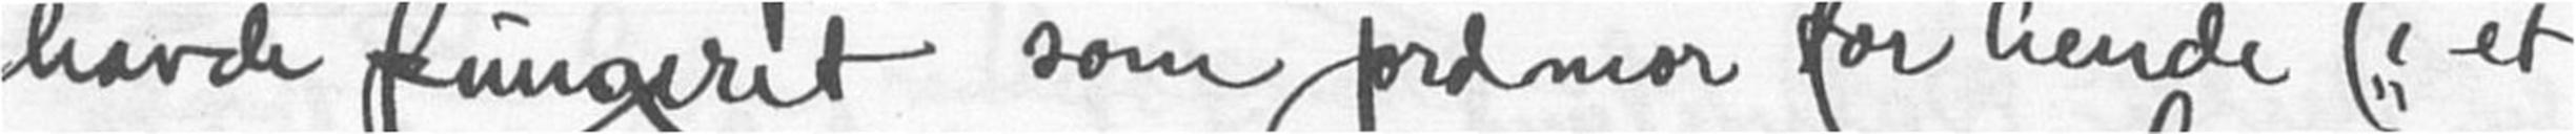havde fungeret som jordmor for hende ("i et
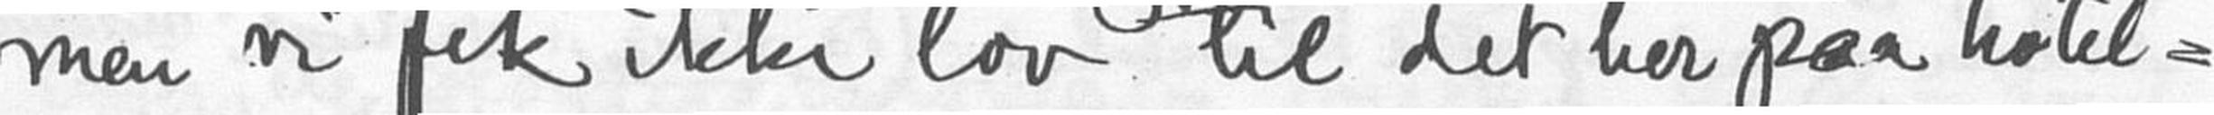men vi fik ikke lov til det her paa hotel-
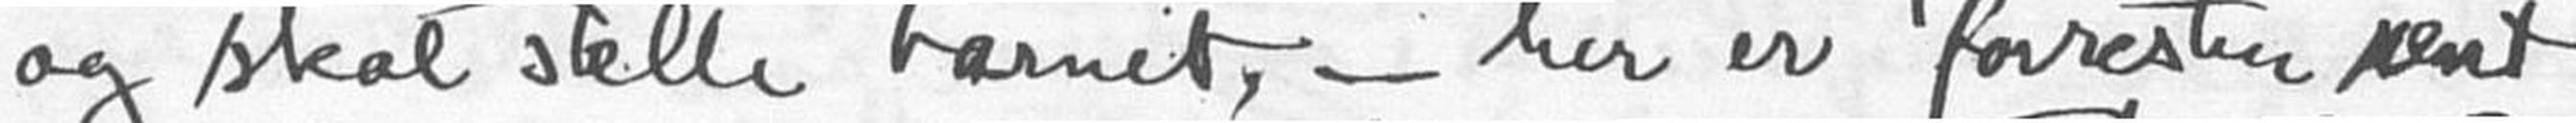og skal stelle barnet, - her er forresten rent
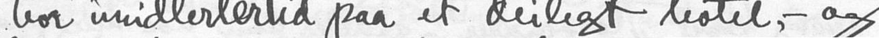bor imidlertid paa et deiligt hotel, - og
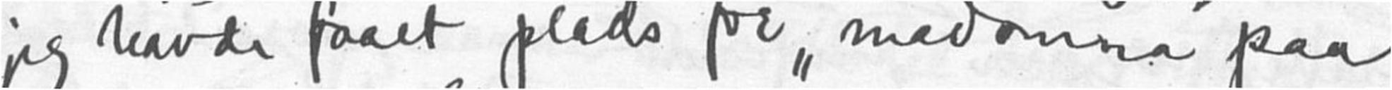jeg havde faaet plads for "Madonna" paa
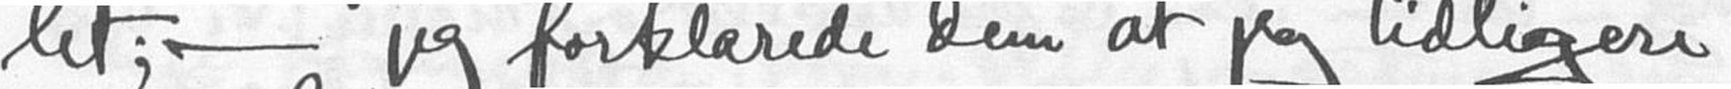let; - jeg forklarede dem at jeg tidligere
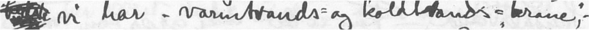vi har varmtvands- og koldtvands-krane; -
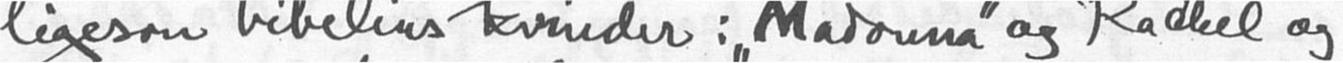ligesom bibelens kvinder: "Madonna" og Rachel og
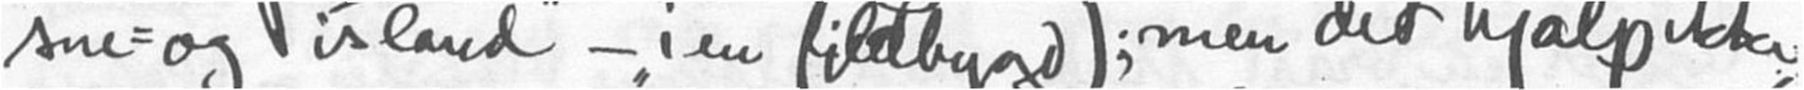sne- og island" -"i en fjeldbygd"); men det hjalp ikke
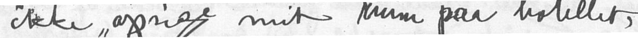ikke "opsige" mit rum paa hotellet,
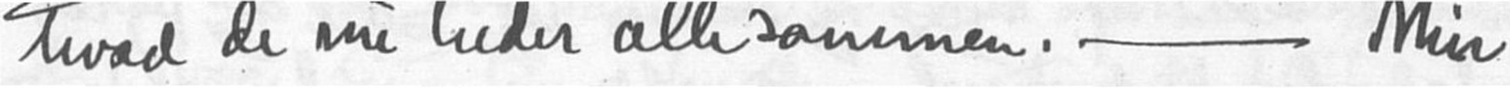hvad de nu heder allesammen, - Min
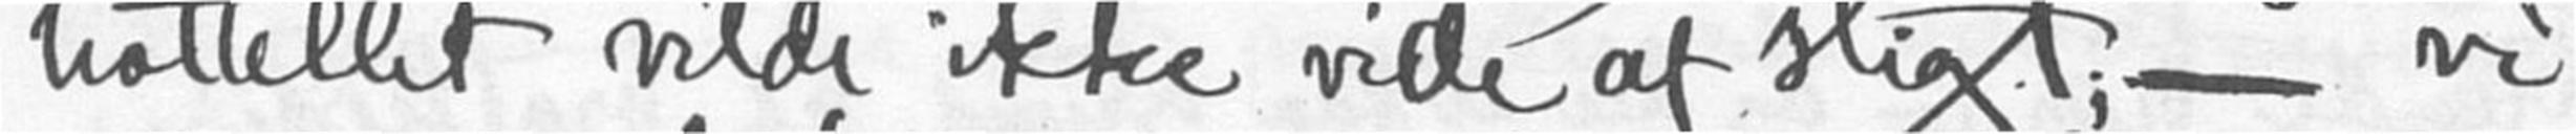hotellet vilde ikke vide af sligt:- vi
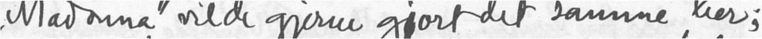"Madonna" vilde gjerne gjort det samme her;
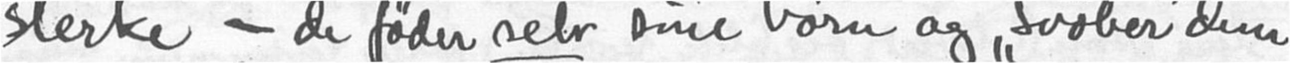sterke - de føder selv sine børn og "svøber" dem
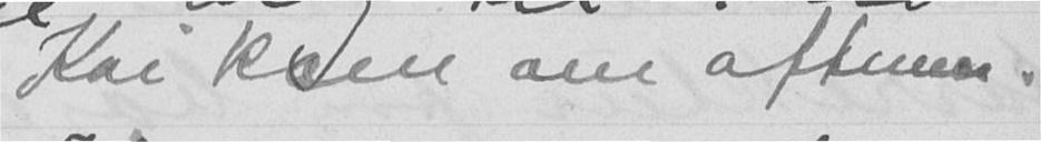Kai kom om aftenen.
